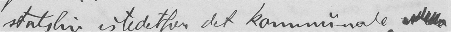statsliv istedetfor det kommunale,
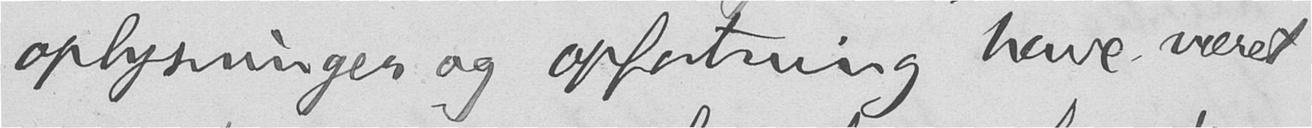oplysninger og opfatning have været
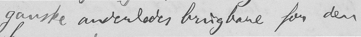ganske anderledes brugbare for den
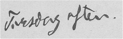Tirsdag aften.
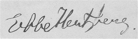Ebbe Hertzberg.
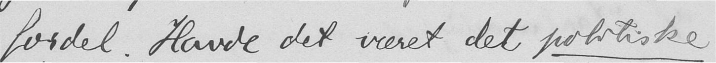fordel. Havde det været det politiske
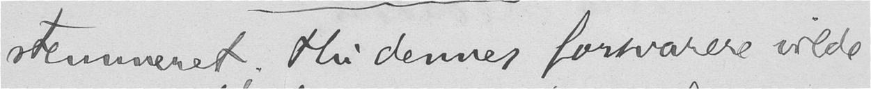stemmeret; thi dennes forsvarere vilde
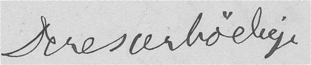Deres ærbødige
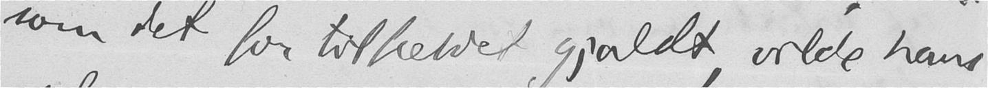som det for tilfældet gjaldt, vilde hans
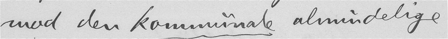mod den kommunale almindelige
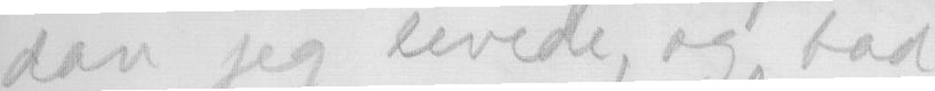dan jeg levede, og bad
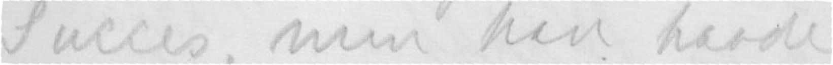Succes, men han havde
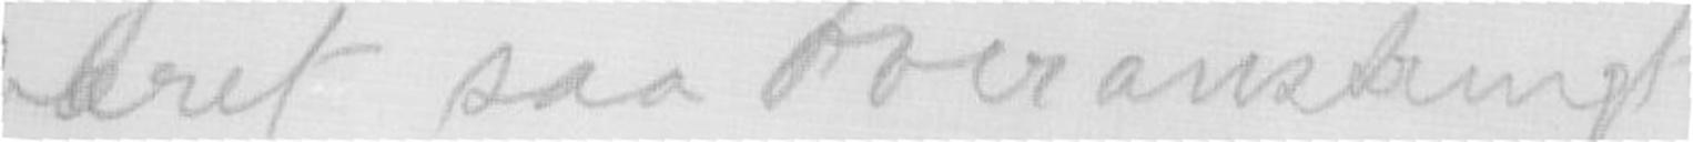været saa overanstrengt
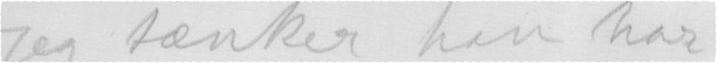Jeg tænker han har
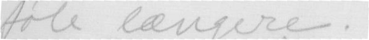føle længere.
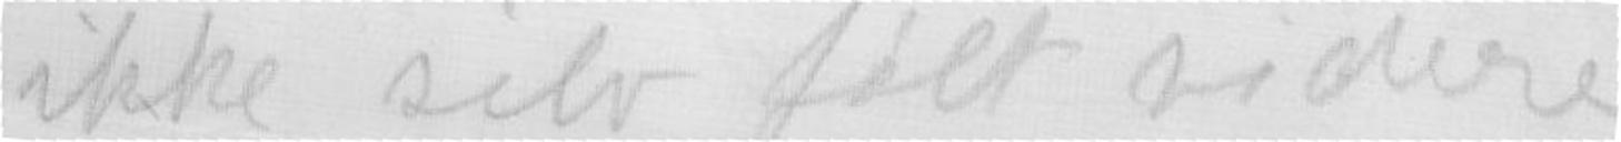ikke selv følt videre
